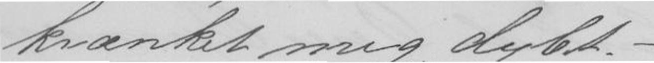krænkt mig dybt. -
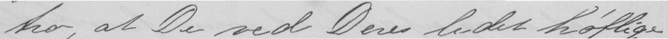end tro, at De ved Deres lidet høflige
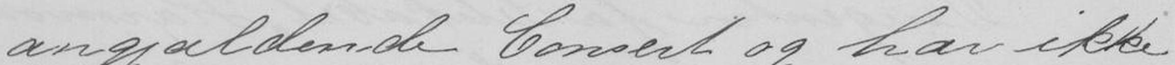angjældende Consert og har ikke
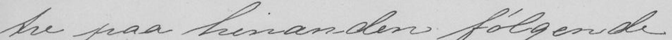tre paa hinanden følgende
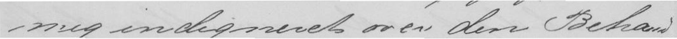mig indigneret over den Behand-
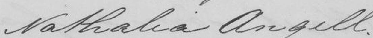Nathalia Angell.
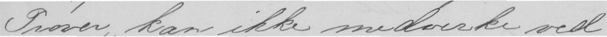Prøver, kan ikke medvirke ved
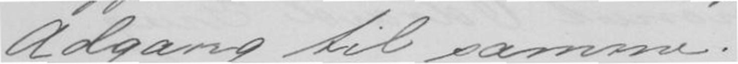Adgang til samme."
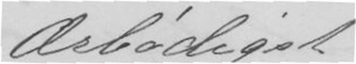Ærbødigst
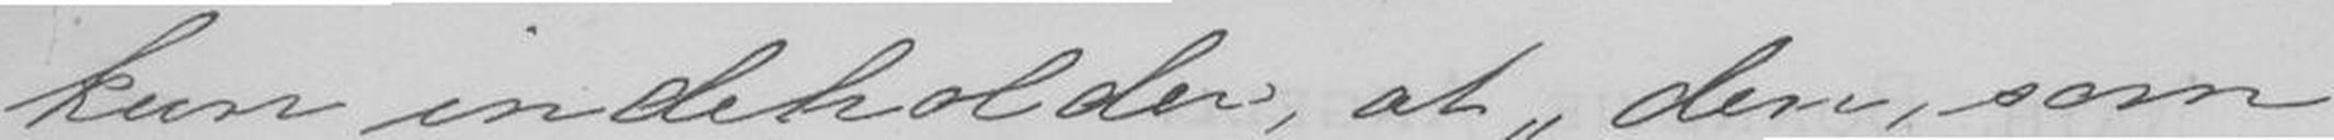kun indeholder, at "den, som
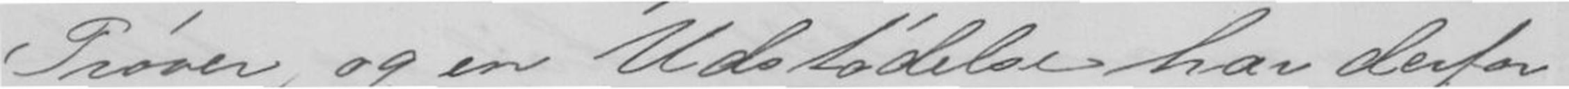Prøver, og en Udstødelse har derfor
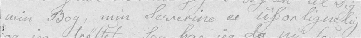min Bog, min Severine er uforlignelig
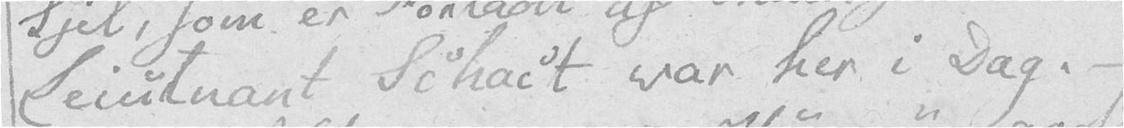Leiutnant Schact var her i Dag. –
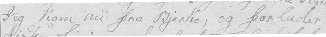Jeg kom nu fra Bjerke, og forlader
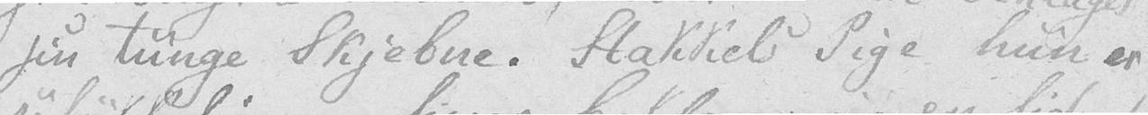sin tunge Skjebne. Stakkels Pige hun er
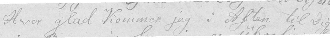Hvor glad Kommer jeg i Aften til Dig
